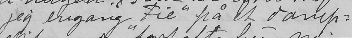jeg engang "Fie" på et damp-
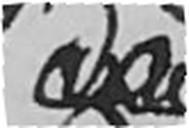Da
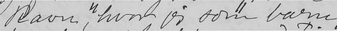Ravn", hvor jeg som barn
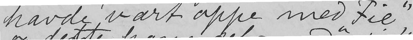havde vært oppe med "Fie",
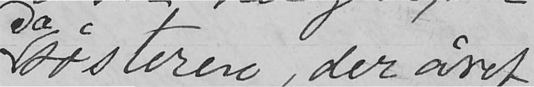søsteren, der året
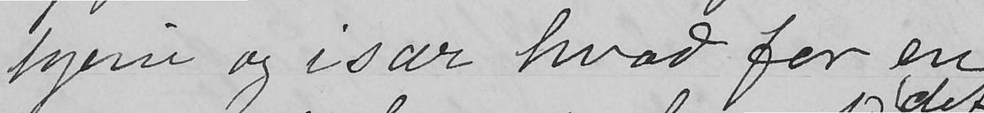hjem og især hvad for en
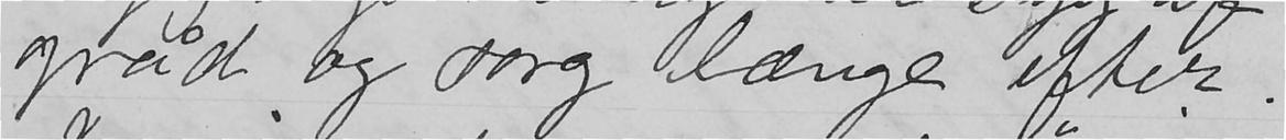gråd og sorg længe efter.
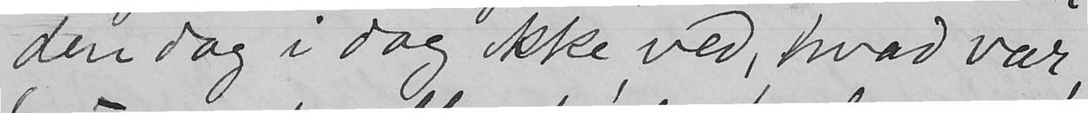den dag i dag ikke ved, hvad var,
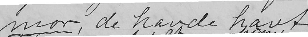mor, de havde havt
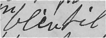blev til
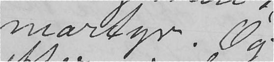martyr. Og
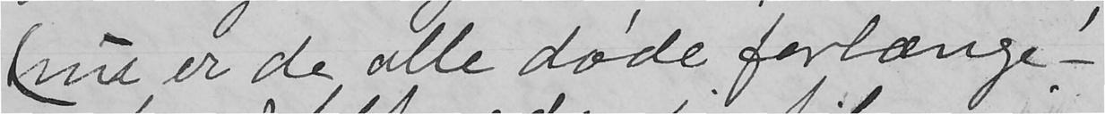(nu er de alle døde forlænge-
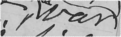var
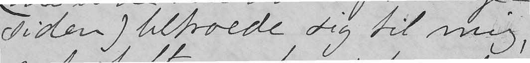siden) betroede sig til mig,
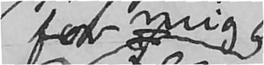for mig.
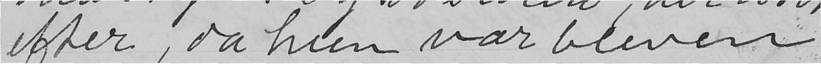efter, da hun var bleven
